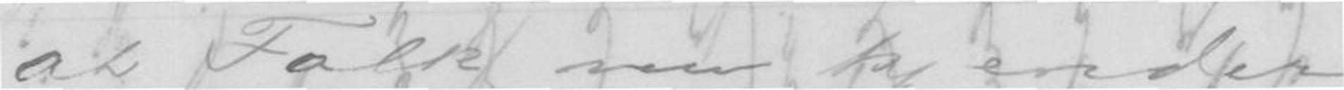at Folk nu kjender
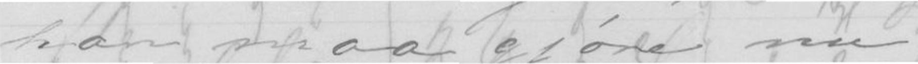han maa gjøre nu.
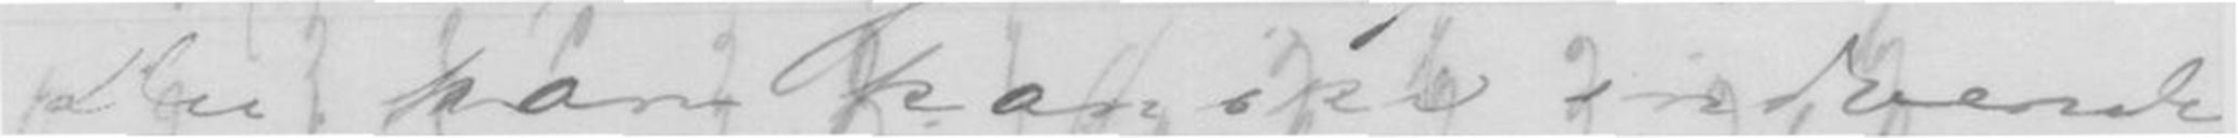Du kan kanske indvende
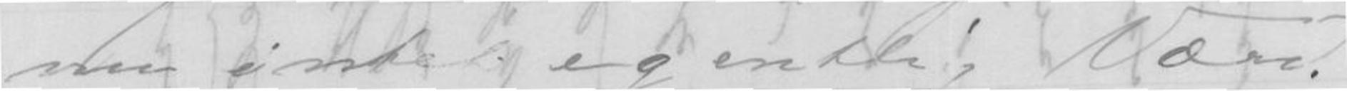nu intet egentlig Værd.
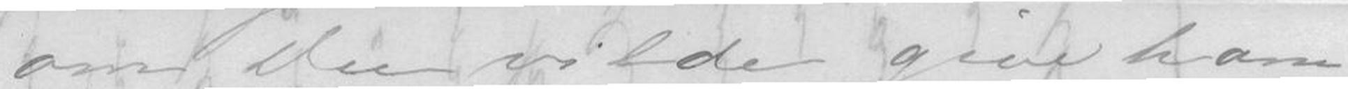om Du vilde give ham
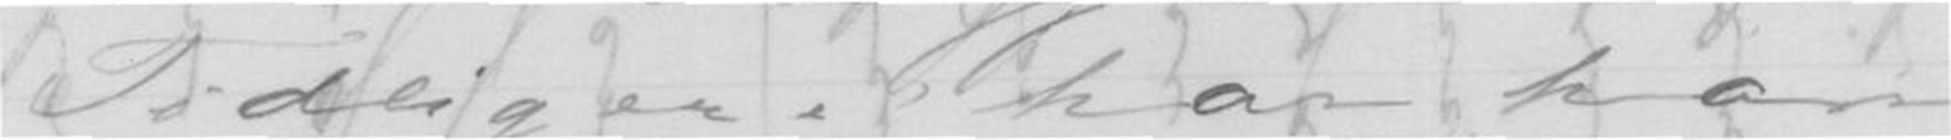Tidligere har han
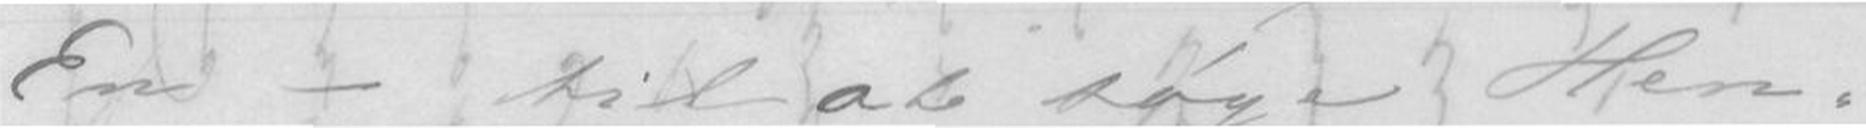En - til at søge Hen-
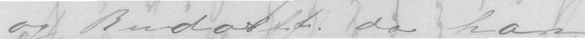og Budorff da han
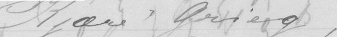Kjære Grieg,
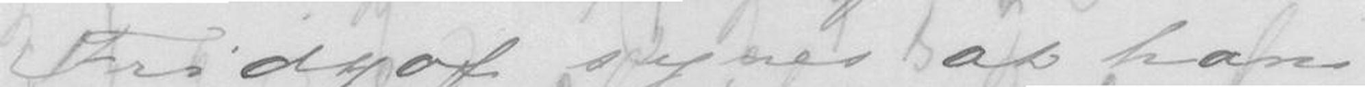Fridtjof synes at han
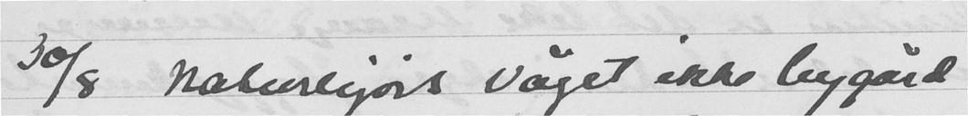30/8 Naturligvis våget ikke Nygård
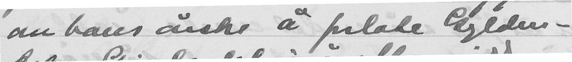om hans ønske å forlate Gylden-
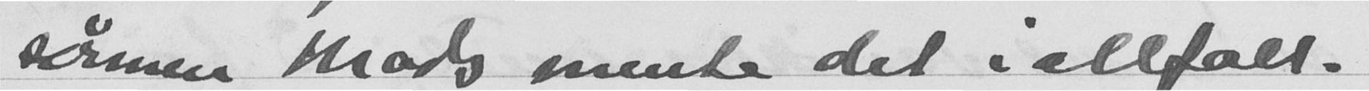sønnen Mads mente det i allfall.
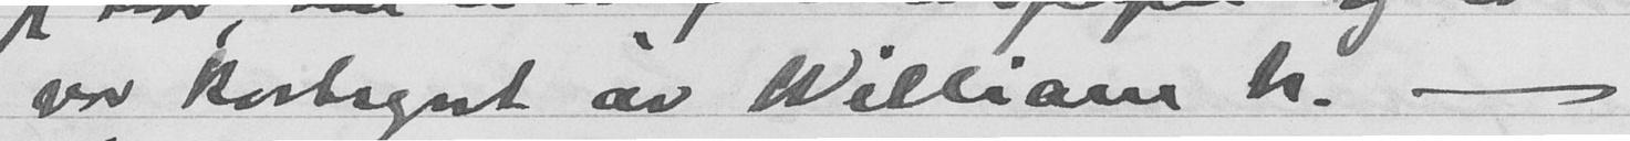var kortsynt av Willian N. -
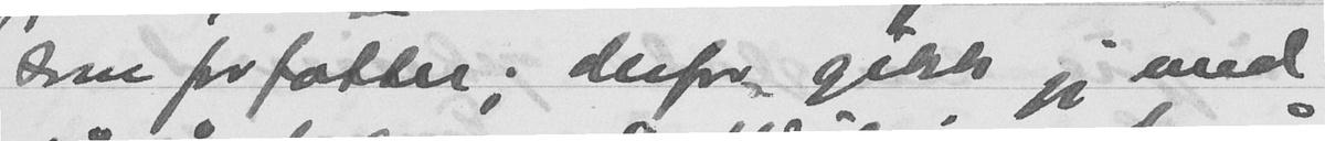som forfatter; derfor gikk jeg med
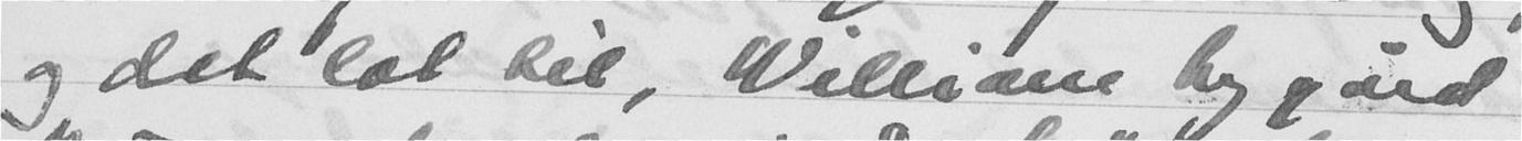og det lot til, William Nygård
